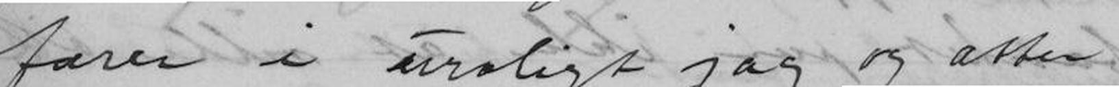farer i uroligt jag og atter
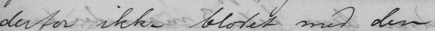derfor ikke blodet med den
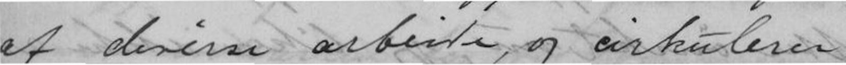af diverse arbeide, og cirkulerer
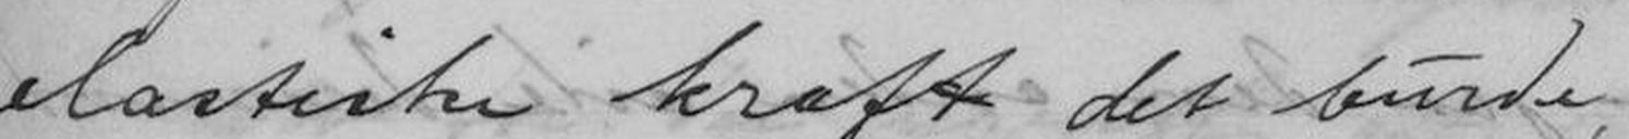elastiske kraft det burde,
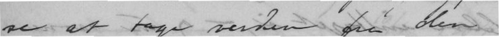se at tage verden for den
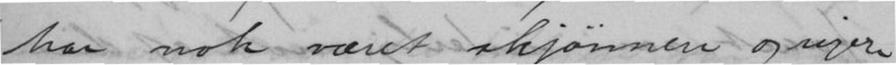har nok været skjønnere og rigere
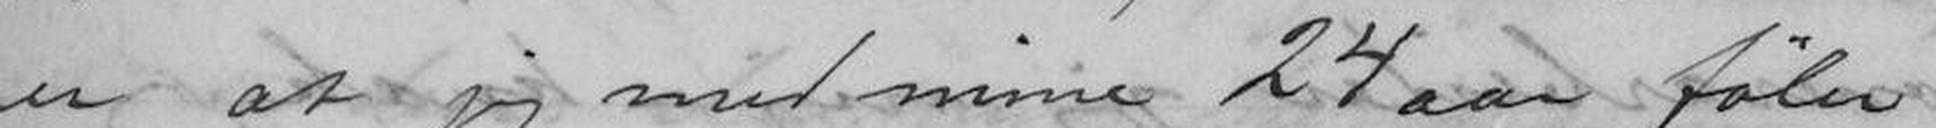er at jeg med mine 24 aar føler
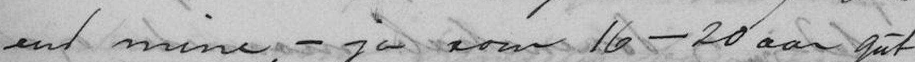end mine, - ja som 16-20 aar gut
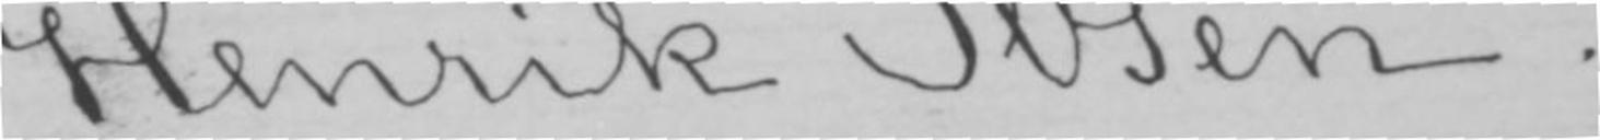Henrik Ibsen.
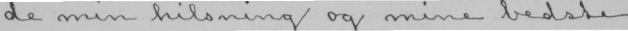de min hilsning og mine bedste
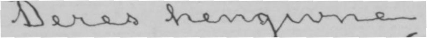Deres hengivne
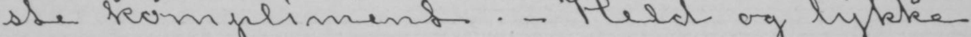ste kompliment.- Held og lykke
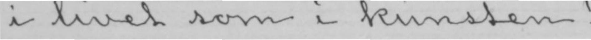i livet som i kunsten!
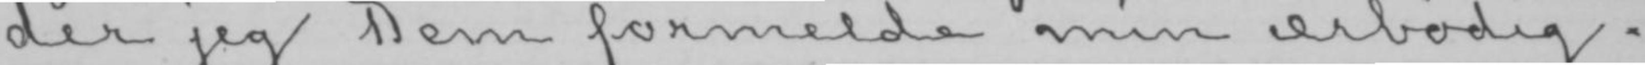der jeg Dem formelde min ærbødig-
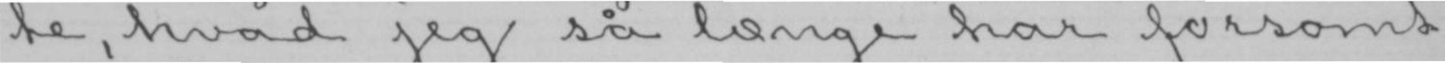te, hvad jeg så længe har forsømt,
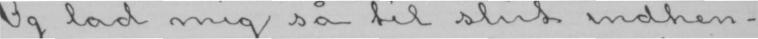Og lad mig så til slut indhen-
